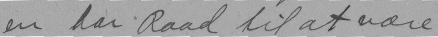en har Raad til at være
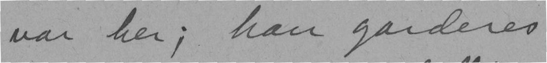var her; han garderes
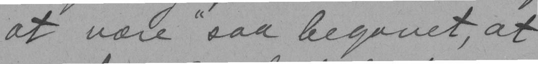at være "saa begavet, at
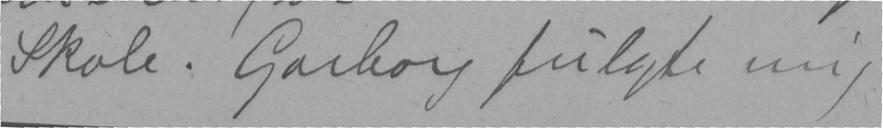Skole. Garborg fulgte mig
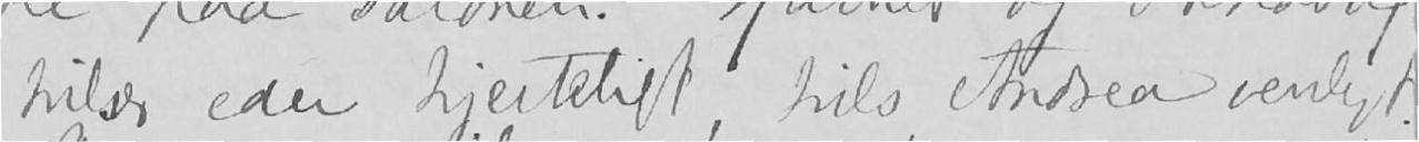hilser eder hjerteligt, hils Andrea venligt.
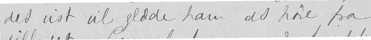det vist vil glæde ham at høre fra
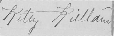Kitty Kielland
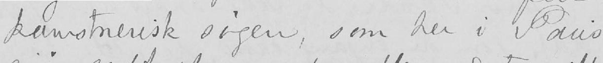kunstnerisk søgen, som her i Paris
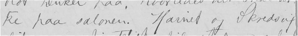ke paa salonen. Harriet og Skredsvig
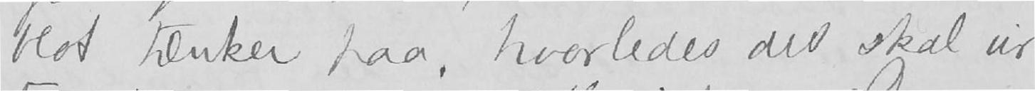blot tænker paa, hvorledes det skal vir-
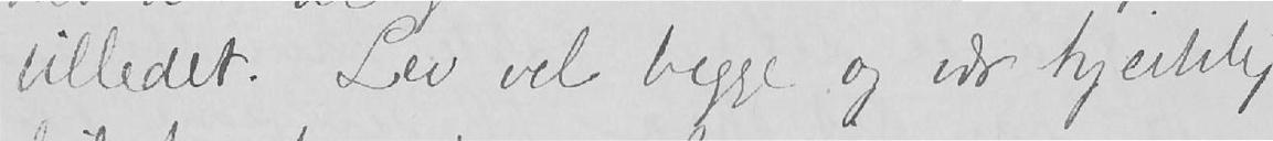billedet. Lev vel begge og vær hjertelig
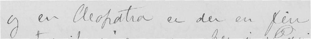og en Cleopatra er der en fin
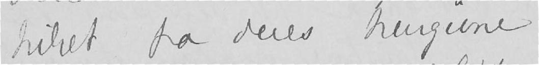hilset fra deres hengivne
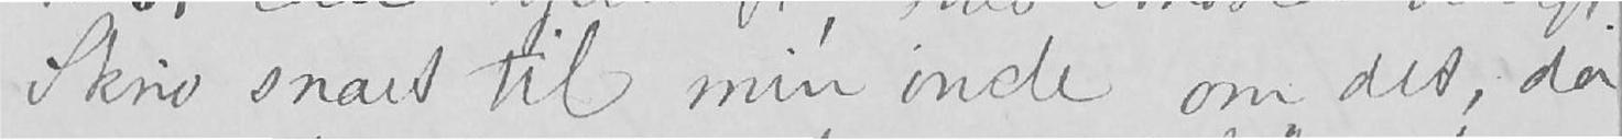Skriv snart til min oncle om det, da
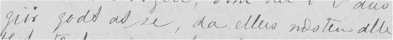giør godt at se, da ellers næsten alle
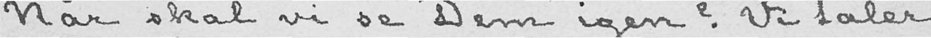Når skal vi se Dem igen? Vi taler
